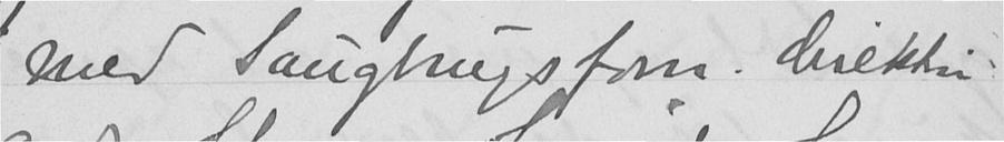med Saugbrugsforn. direktør
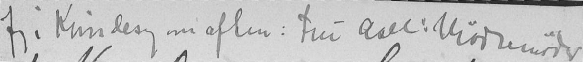Jeg i Kvindesag om aften: Fru Aall i Mødremøde
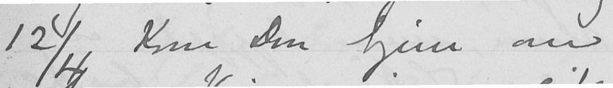12/4 Kom Don hjem om
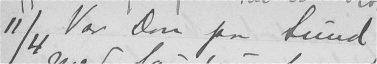11/4 Var Don paa Sund
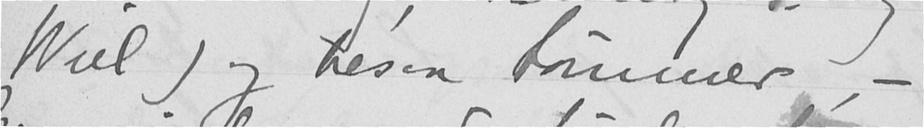Wiel) og tesen tømmer, -
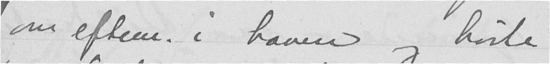om efterm. i haven og hørte
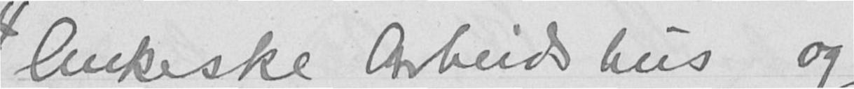Ankerske Arbeidshus og
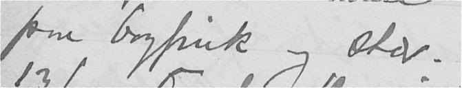paa bogfink og stær.
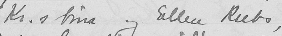Kr.s børn og Ellen Rubo,
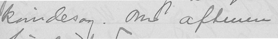kvindesag. Om aftenen
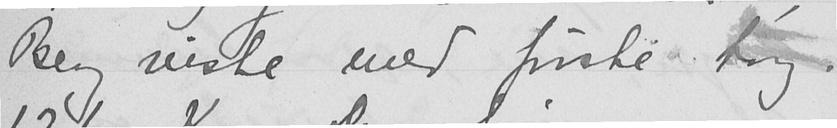Berg reiste med første tog.
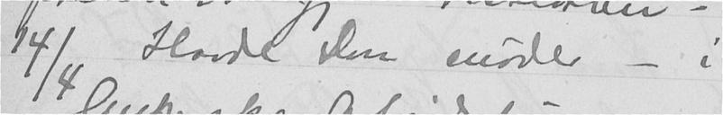14/4. Havde Don møder - i
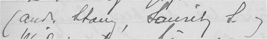(and. Stang, Lauritz S. og
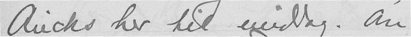Riecks her til middag. Om
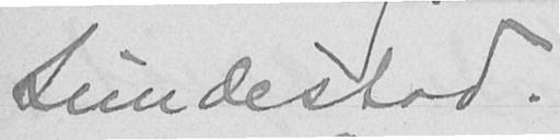Lundestad.
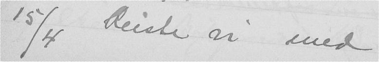15/4 Reiste vi med
